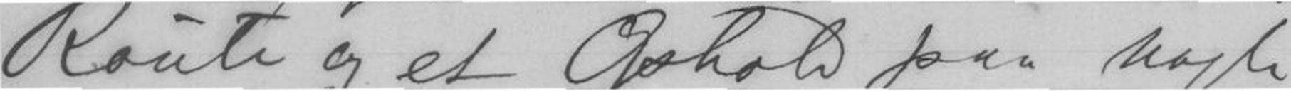Route og et Ophold paa nogle
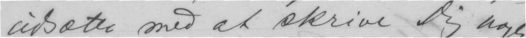udsætte med at skrive Dig nogle
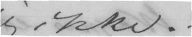ikke.-
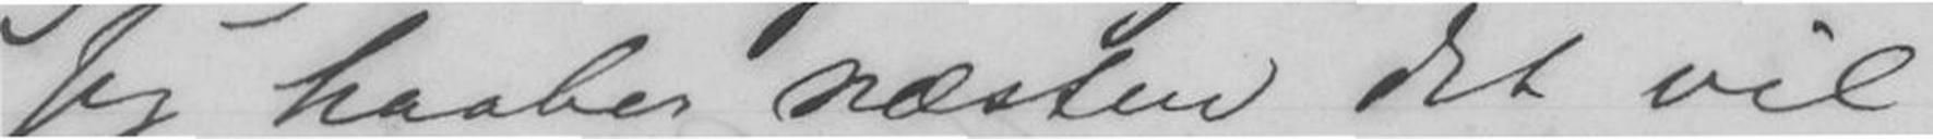Jeg haaber næsten det vil
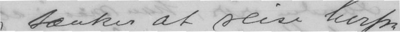Jeg tænker at reise herfra
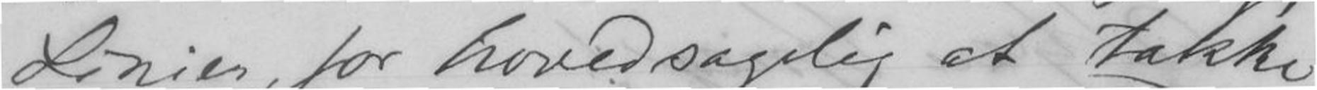Linier, for hovedsagelig at takke
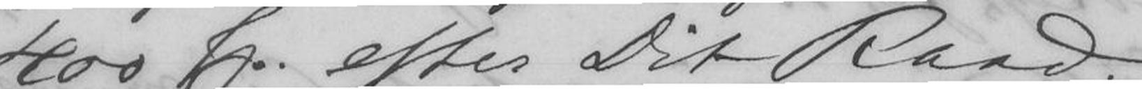400 Sp. efter Dit Raad.
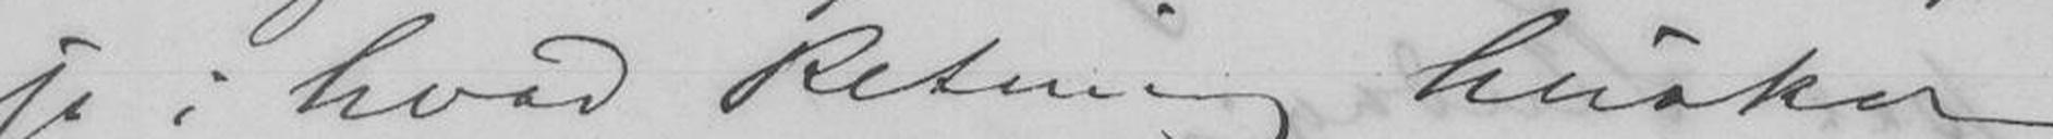ja i hvad Retning husker jeg
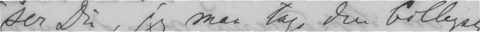ser Du, jeg maa tage den billigste
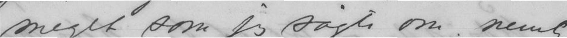meget som jeg søgte om nemlig
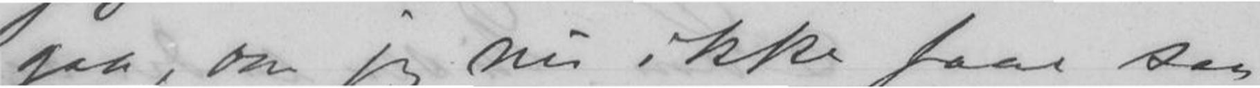gaa, om jeg nu ikke faar saa
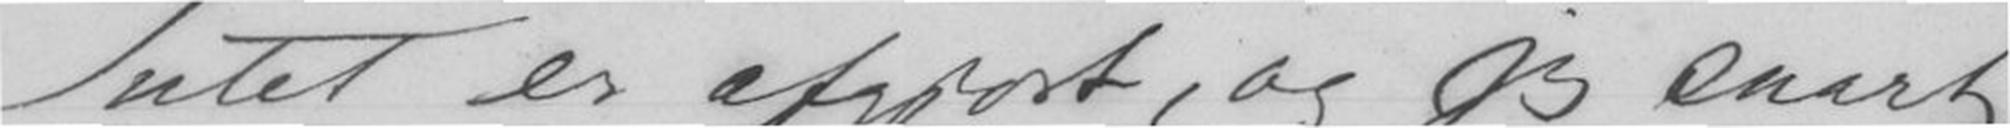Intet er afgjort, og jeg snart
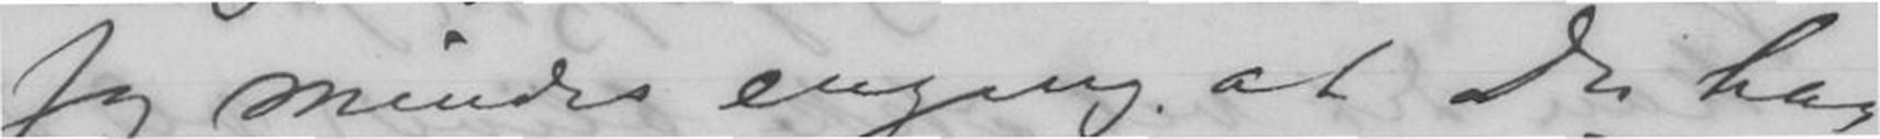Jeg mindes engang at Du har
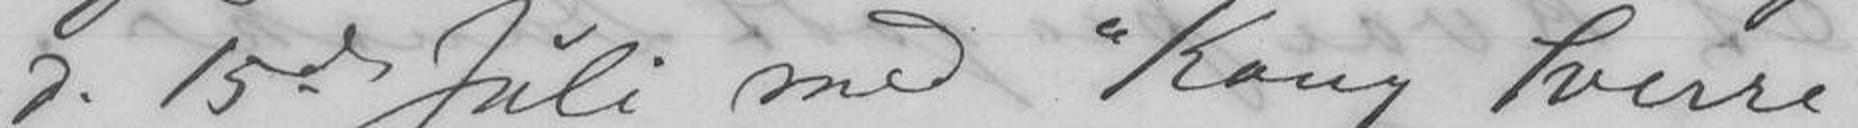d. 15de Juli med "Kong Sverre"
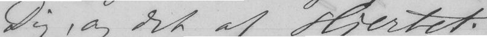Dig, og det af Hjertet.
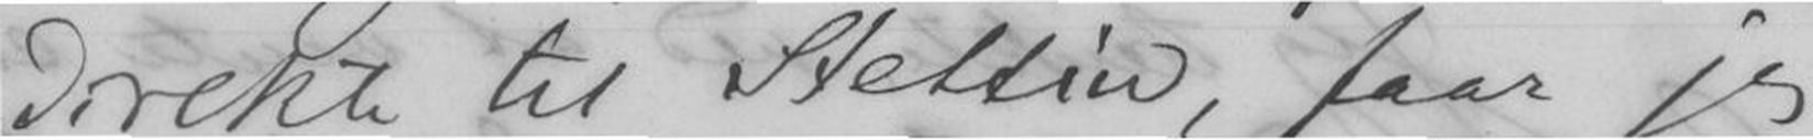Direkte til Sleetin, faar jeg
